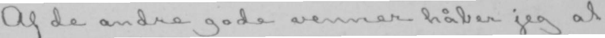Af de andre gode venner håber jeg at
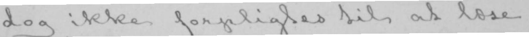dog ikke forpligtes til at læse,
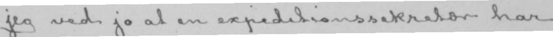jeg ved jo at en expeditionssekretær har
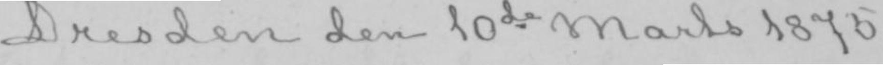Dresden den 10de Marts 1875.
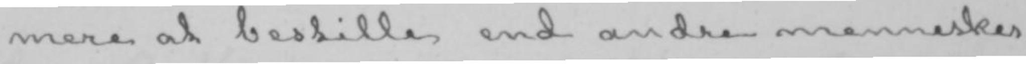mere at bestille end andre mennesker.
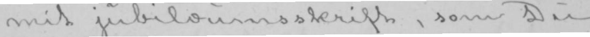mit jubilæumsskrift, som Du
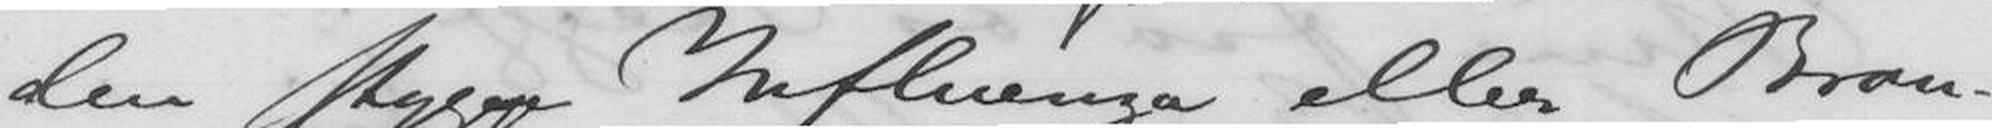den stygge Indfluenza eller Bron-
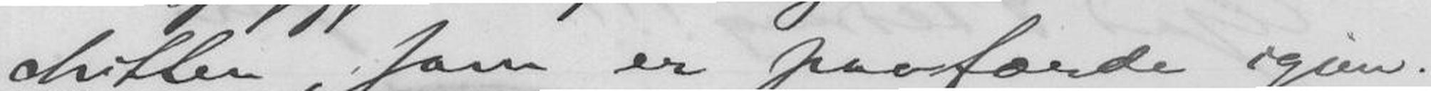chitten, som er paafærde igjen.
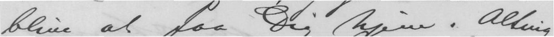blive at faa Dig hjem. Alting
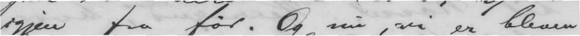igjen fra før. Og nu, vi er bleven
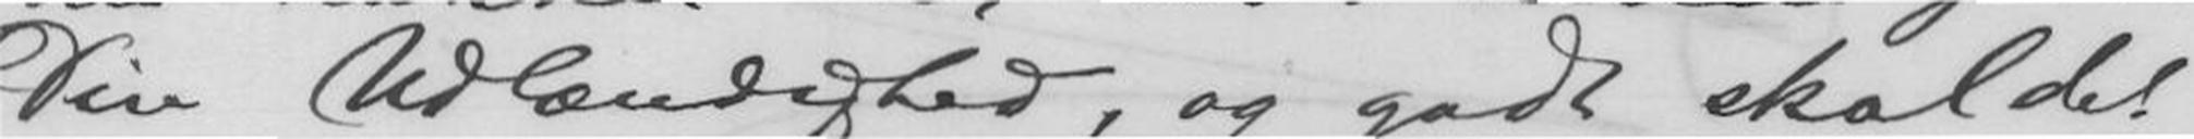Din Udlændighed, og godt skal det
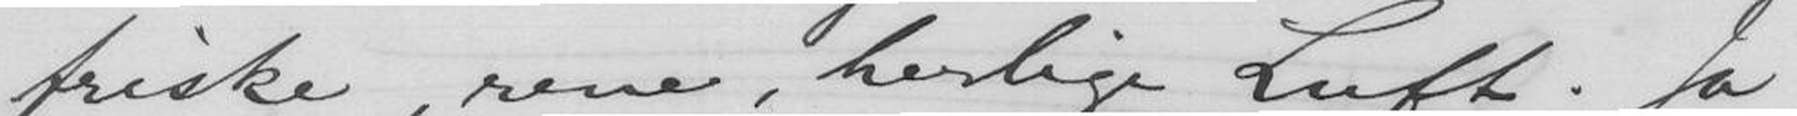friske, rene, herlige Luft. Ja
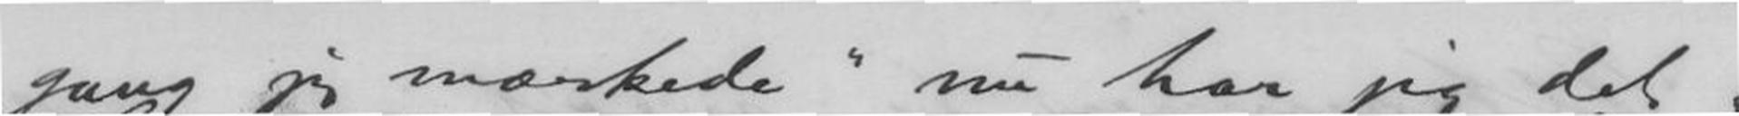gang jeg mærkede "nu har jeg det."
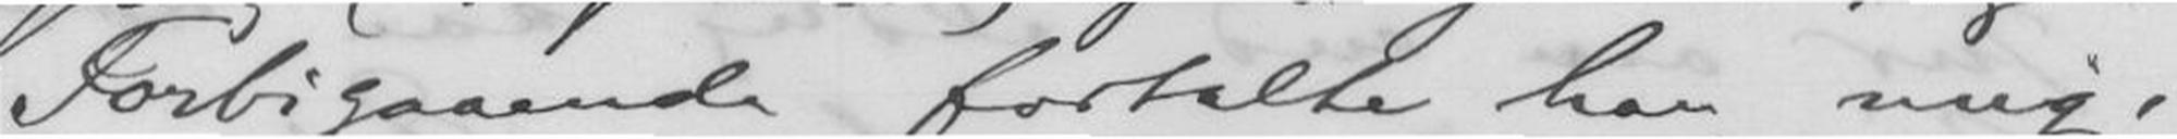Forbigaaende fortalte han mig,
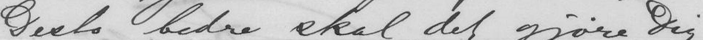Desto bedre skal det gjøre Dig
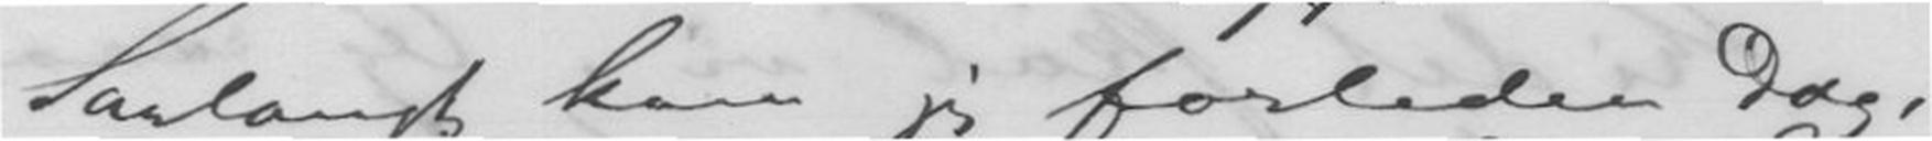Saalangt kom jeg forleden Dag,
